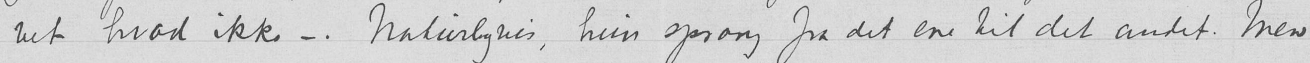vet hvad ikke –. Naturligvis, hun sprang fra det ene til det andet. Men
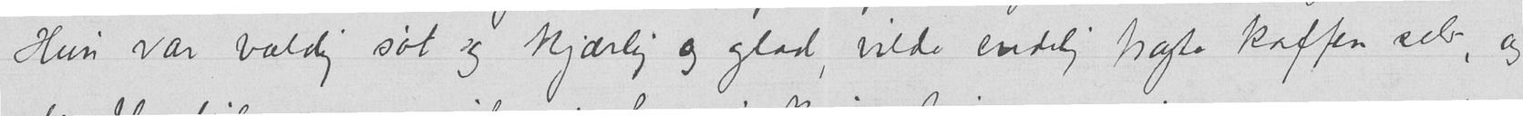Hun var vældig søt og kjærlig og glad, vilde endelig tragte kaffen selv, og
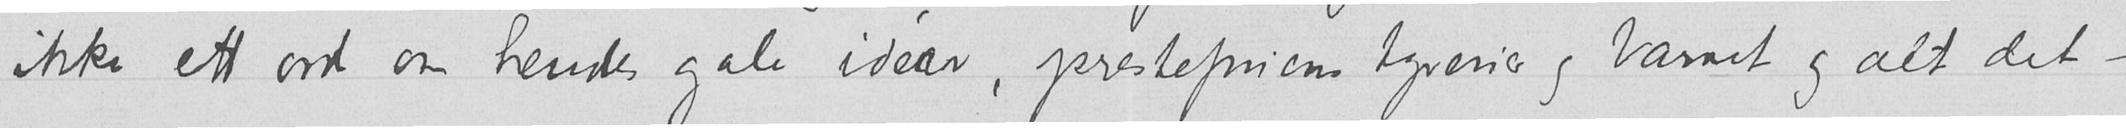ikke ett ord om hendes gale idèer, prestefruens tyverier og barnet og alt det –.
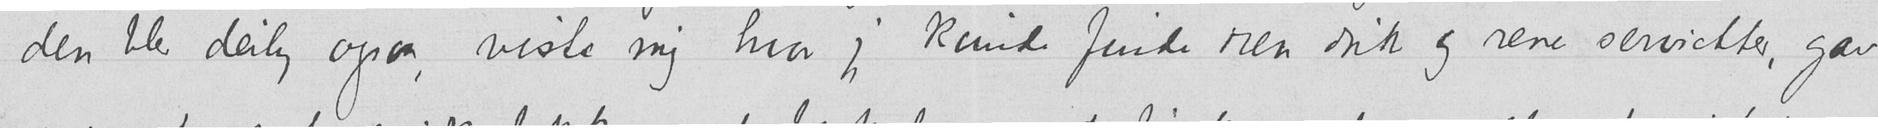den ble deilig ogsaa, viste mig hvor jeg kunde finde ren duk og rene servietter, gav
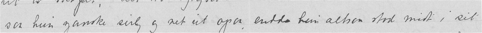saa hun ganske sirlig og net ut ogsaa, endda hun altsaa stod midt i sit
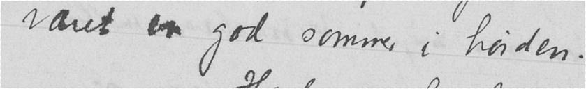været en god sommer i høiden.
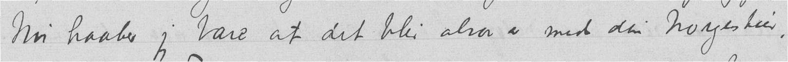Nu haaber jeg bare at det blir alvor av med din Norgestur,
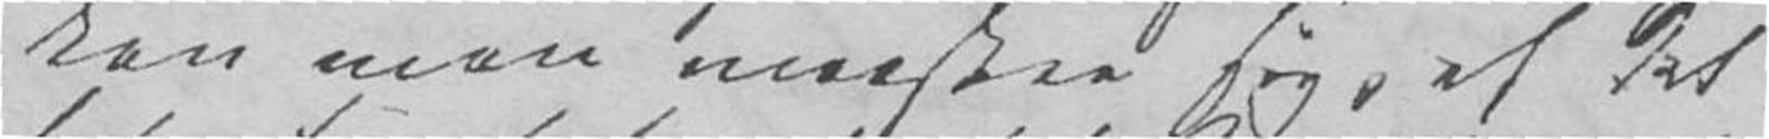kan man maaskee sige at det
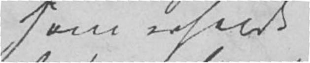som erholdt
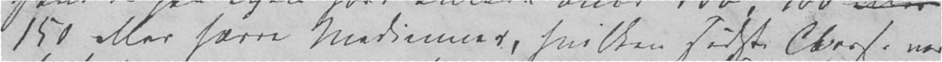150 eller færre Medimner, hvilken sidste Classe var
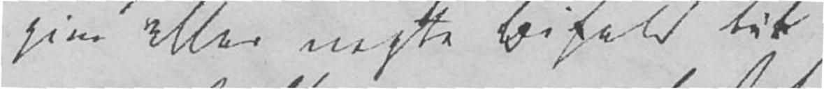give eller nægte Bifald til
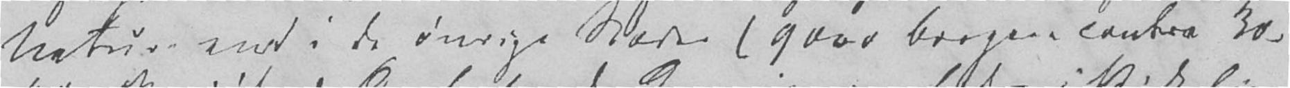Natur end i de øvrige Stæder (9000 Borgere contra 30-
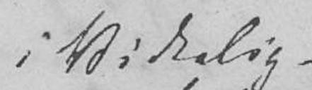i Virkelig-
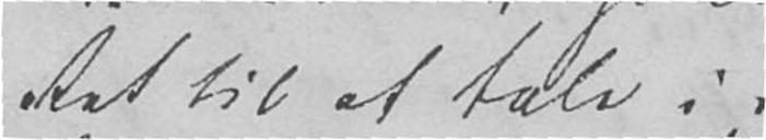Ret til at tale i
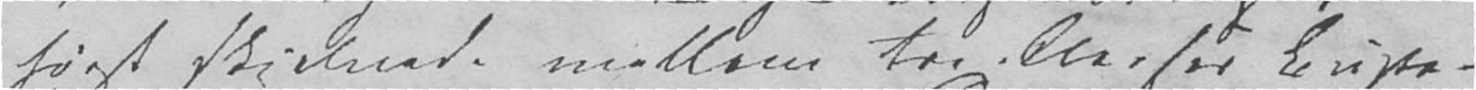først skjelnede mellom tre Classer kuste-
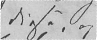disse, og
